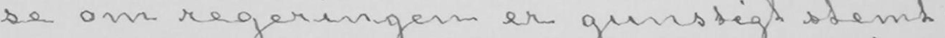se om regeringen er gunstigt stemt
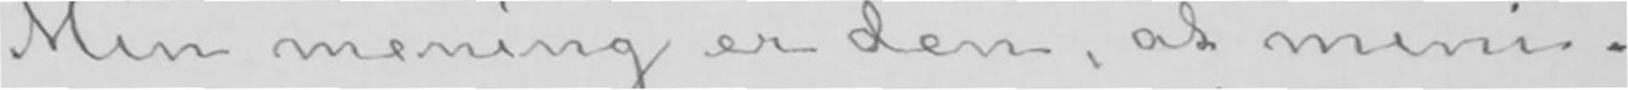Min mening er den, at mini-
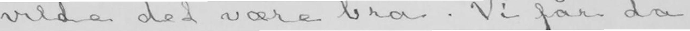vilde det være bra. Vi får da
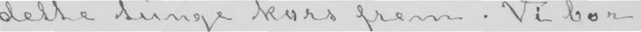dette tunge kors frem. Vi bør
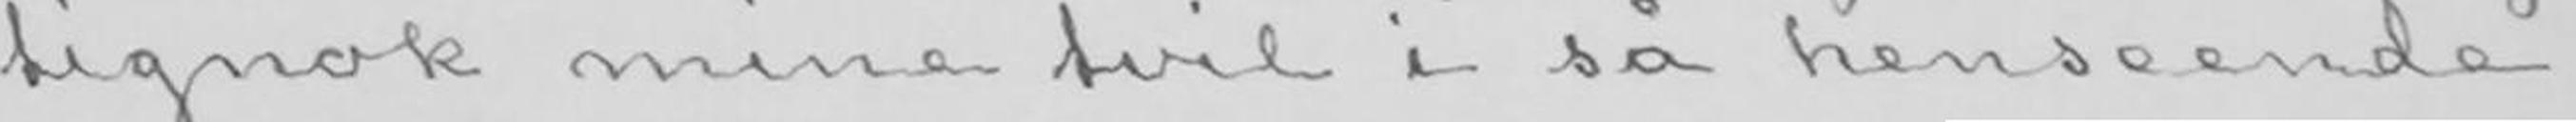tignok mine tvil i så henseende.
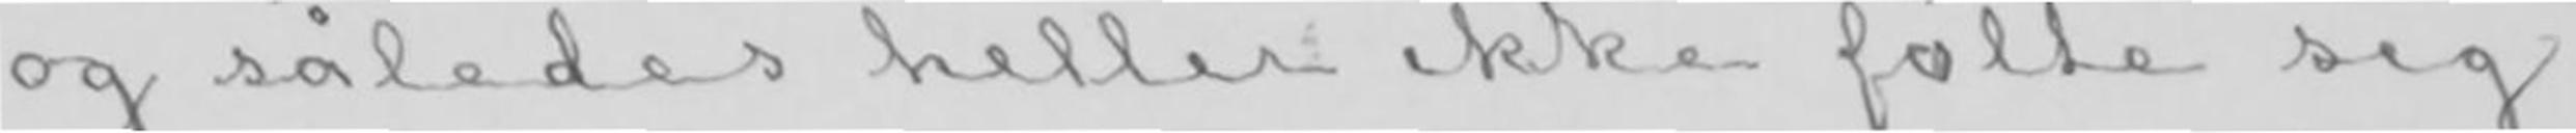og således heller ikke følte sig
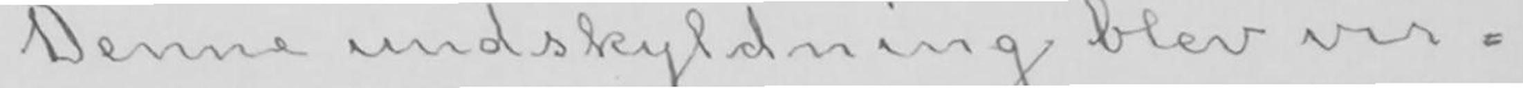Denne undskyldning blev vir-
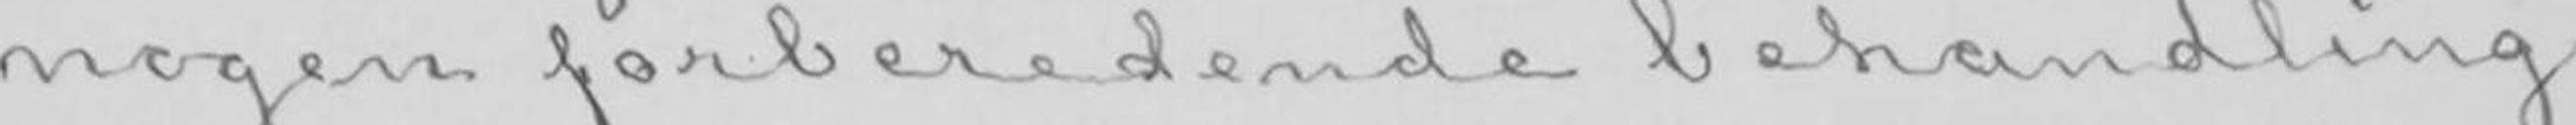nogen forberedende behandling
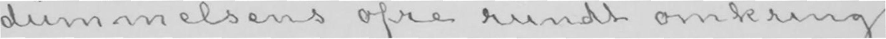dummelsens ofre rundt omkring
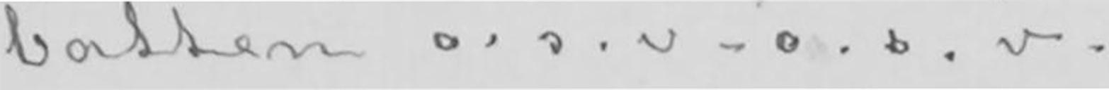batten o. s. v.- o. s. v.
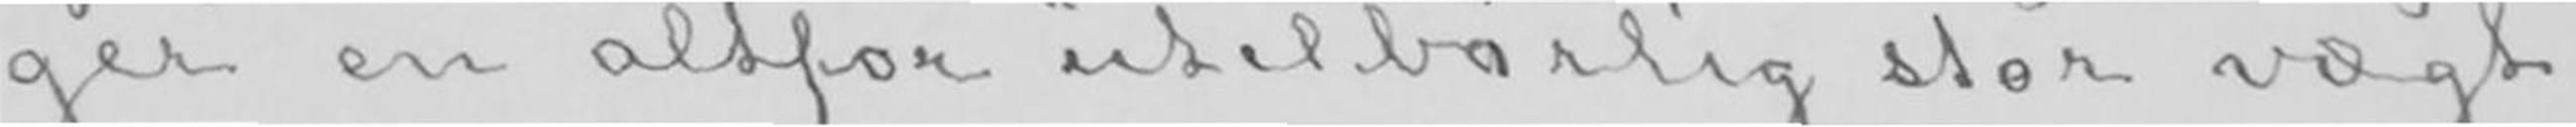ger en altfor utilbørlig stor vægt
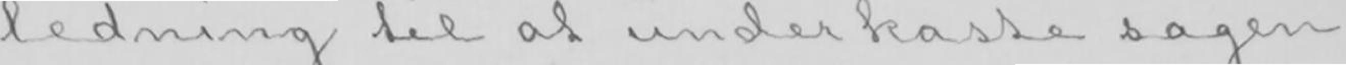ledning til at underkaste sagen
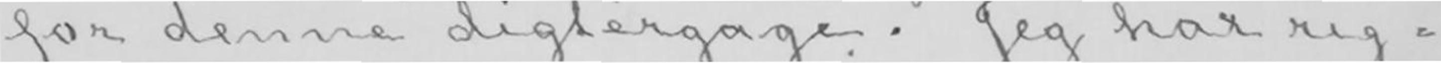for denne digtergage. Jeg har rig-
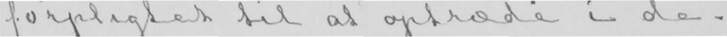forpligtet til at optræde i de-
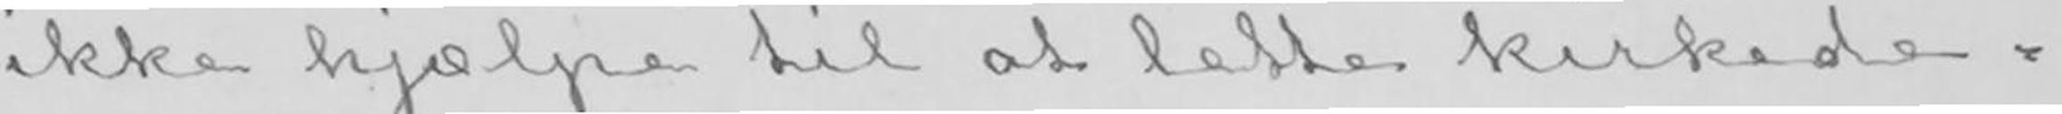ikke hjælpe til at lette kirkede-
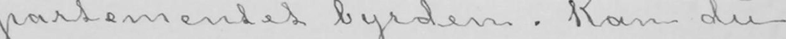partementet byrden. Kan du
